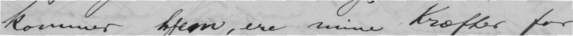kommer hjem, ere mine Kræfter for
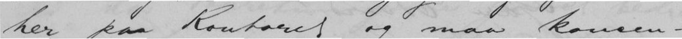her paa Kontoret og maa konsen-
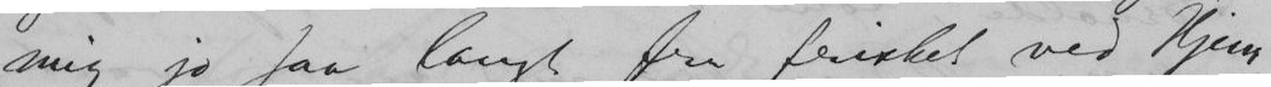mig jo saa langt fra frisket ved Hjem-
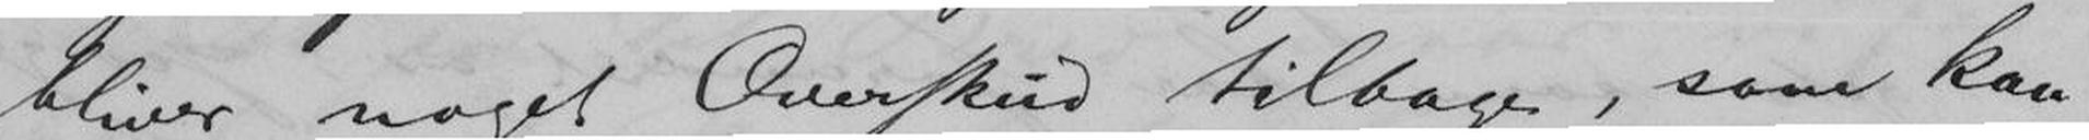bliver noget Overskud tilbage, som kan
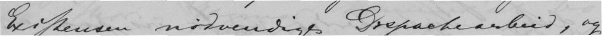Existensen nødvendige Dispachearbeid, og
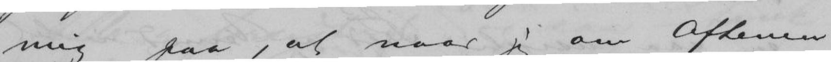mig paa, at naar jeg om Aftenen
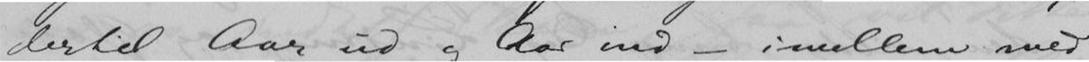dertil Aar ud og Aar ind - imellem med
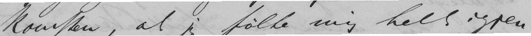komsten, at jeg følte mig helt igjen-
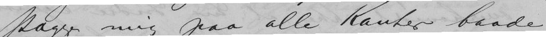stagge mig paa alle Kanter baade
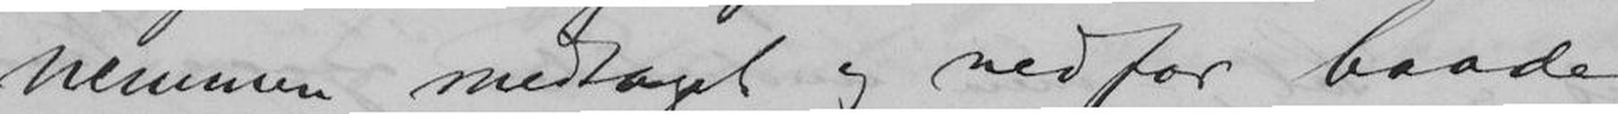nemmen medtaget og nedfor baade
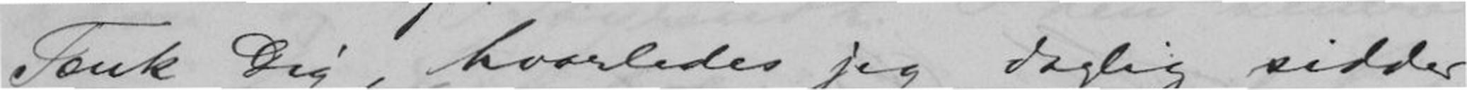Tænk Dig, hvorledes jeg daglig sidder
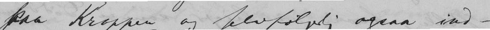paa Kroppen og selvfølgelig ogsaa ind-
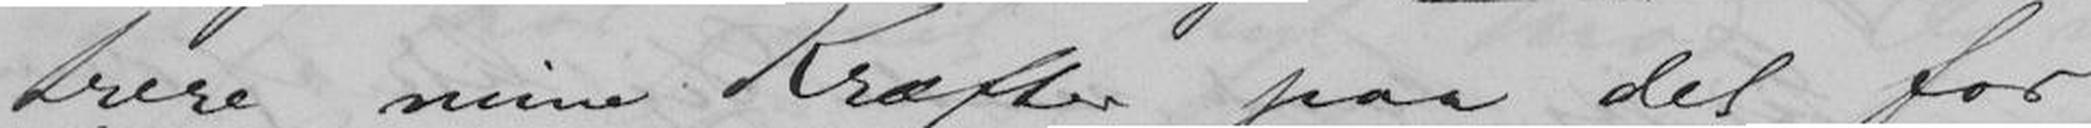trere mine Kræfter paa det for
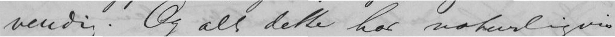vendig. Og alt dette har naturligvis
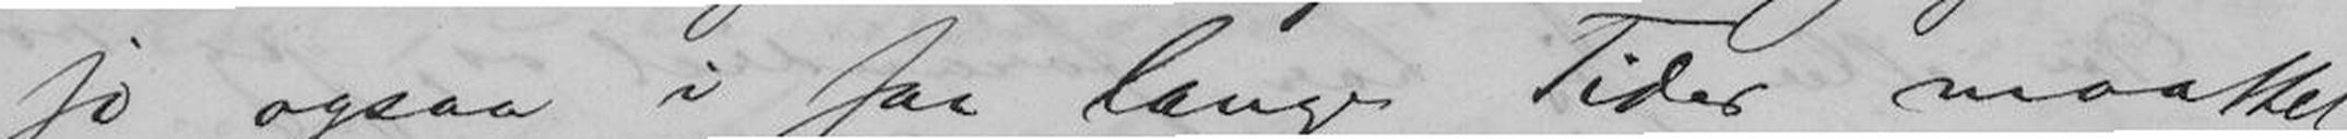jo ogsaa i saa lange Tider maattet
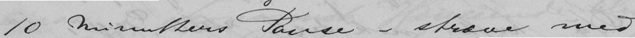10 Minutters Pause - stræve med
